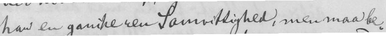har en ganske ren Samvittighed, men maa be-
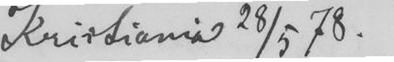Kristiania 28/5 78.
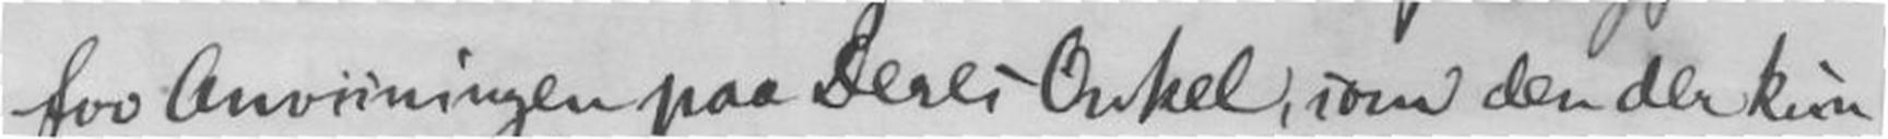for Anvisningen paa Deres Onkel, som den der kun
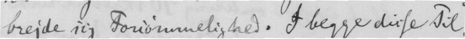brejde sig Forsømmelighed. I begge disse Til-
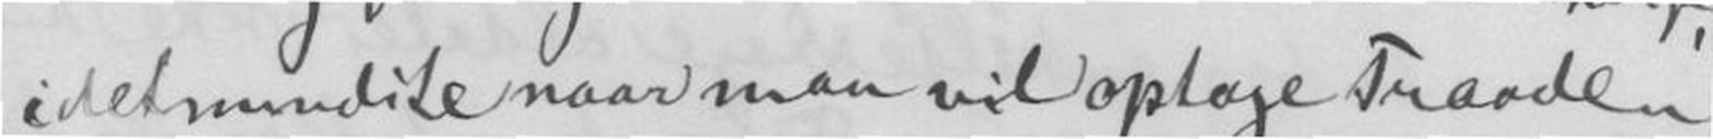i dat mindste naar man vil optage Traaden
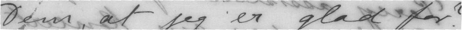Dem, at jeg er glad for?
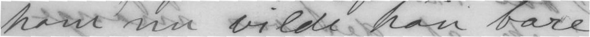ham nu vilde han bare
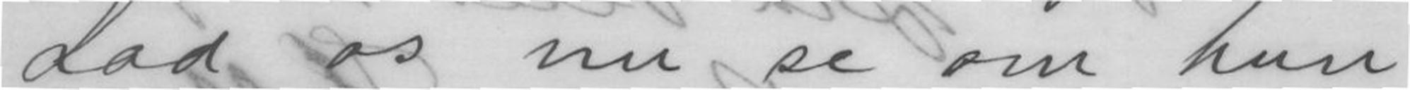Lad os nu se om hun
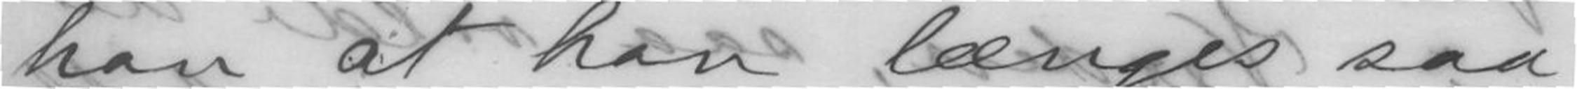han at han længes saa
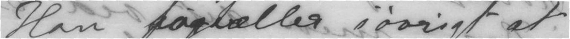Han forjtæller iøvrigt at
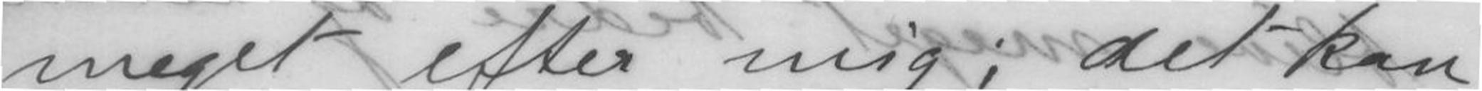meget efter mig; det kan
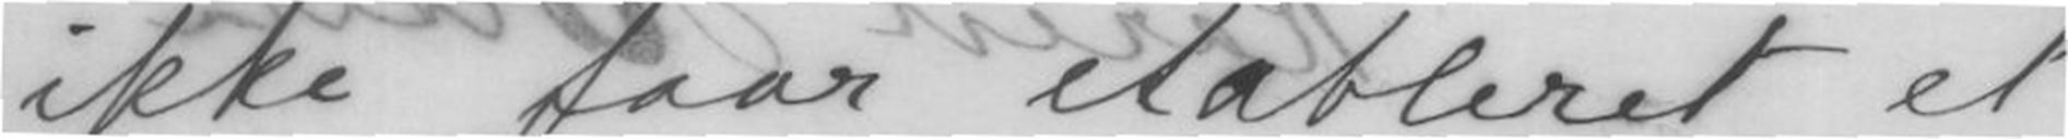ikke faar etablert et
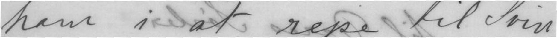ham i at rejse til Svin
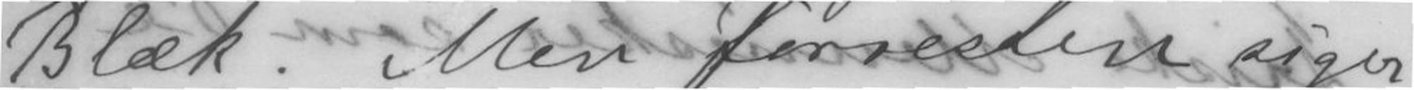Blæk. Men forresten siger
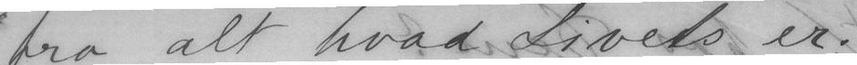fra alt hvad Livets er;
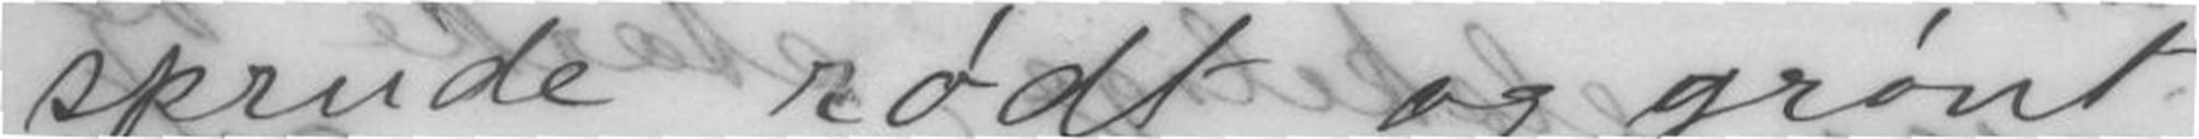sprude rødt og grønt
